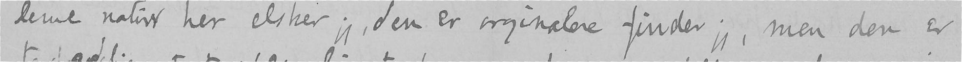denne natur her elsker jeg, den er orginalere finder jeg, men den er
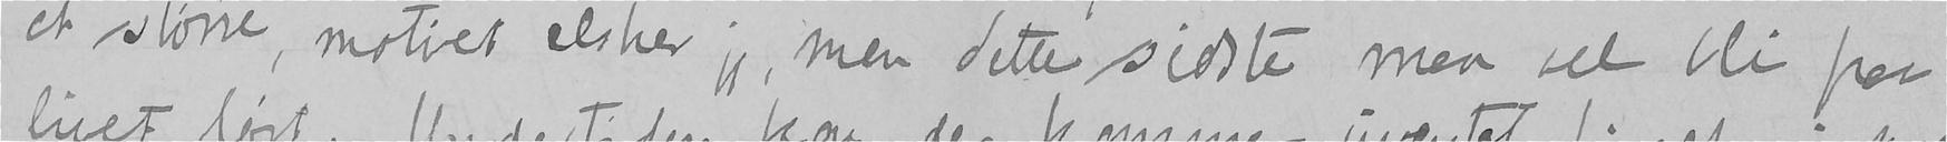et større, motivet elsker jeg, men dette sidste maa vel bli paa
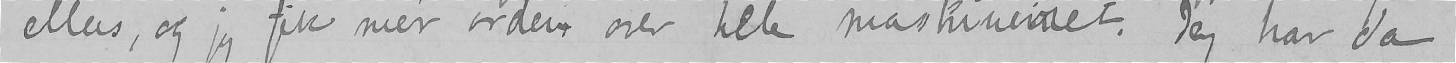ellers, og jeg fik mer orden over hele maskineriet. Jeg har da
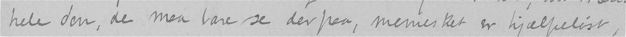hele den, de maa bare se derpaa, mennesket er hjælpeløst,
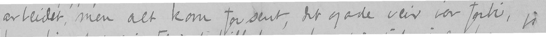arbeidet, men alt kom for sent, det gode veir var forbi, jeg
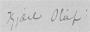Kjære Oluf!
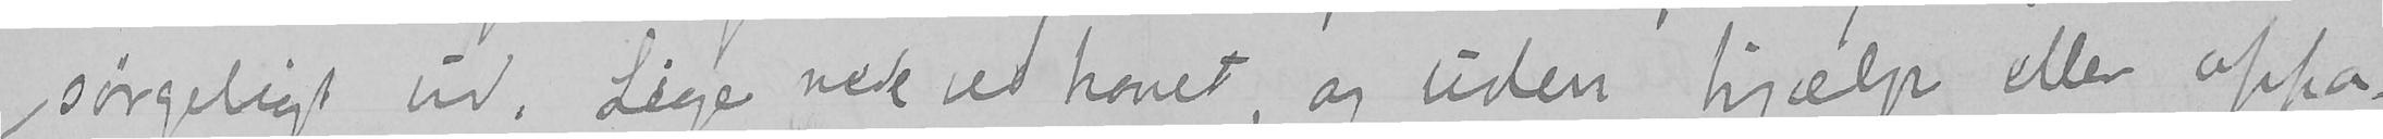sørgeligt ud. Lige ned ved havet og uden hjælp eller appa-
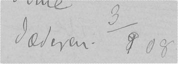Jæderen 3/9 08
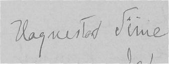Hognestad Time
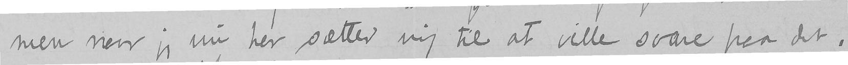men naar jeg nu her sætter mig til at ville svare paa det,
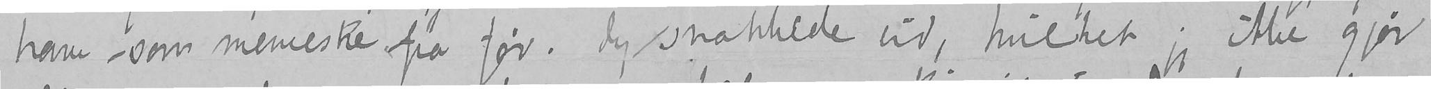ham som menneske fra før. Jeg snakkede ud, hvilket jeg ikke gjør
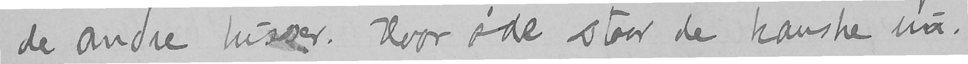de andre huser. Hvor øde staar de kanske nu.
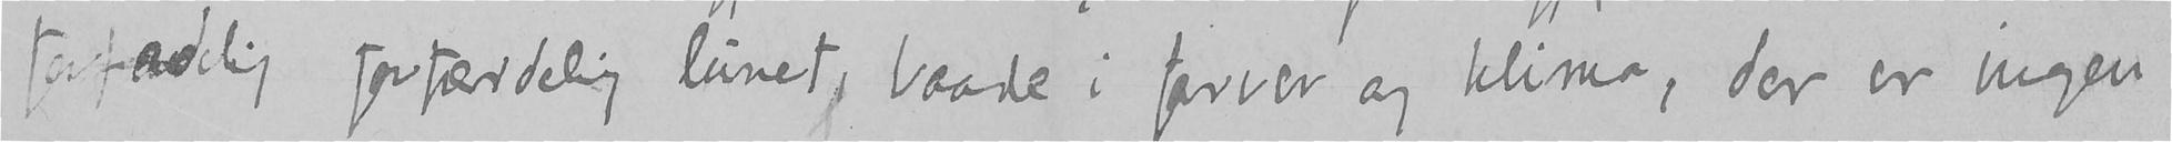forfadelig forfærdelig lunet, baade i farver og klima, der er ingen
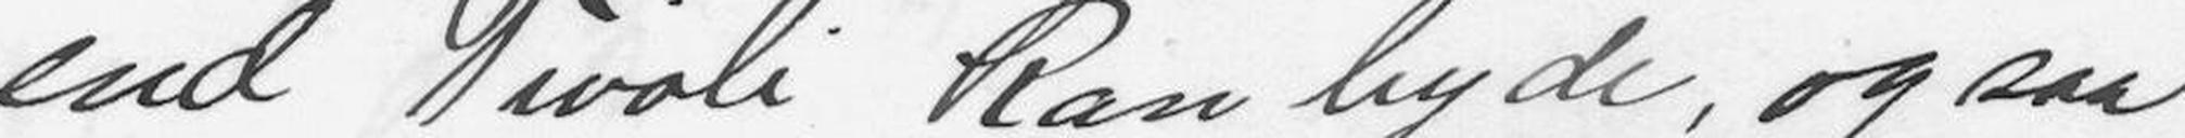end Tivoli kan byde, og saa
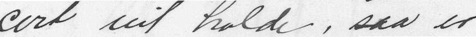cert vil holde, saa er
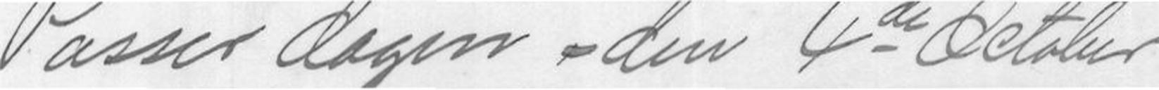Passer dagen den 4de October
